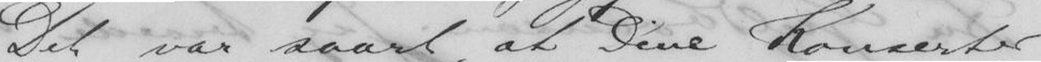Det var saart, at Dine Konserter
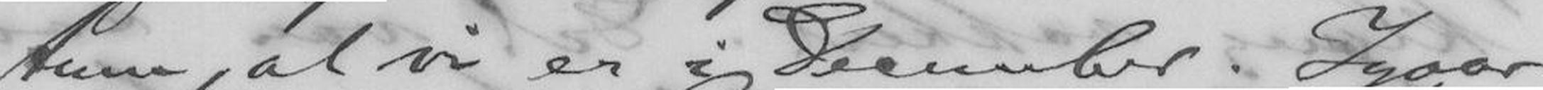tum, at vi er i December. Igaar
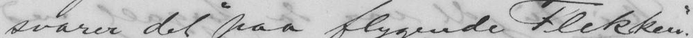svarer det "paa flygende Flekken".
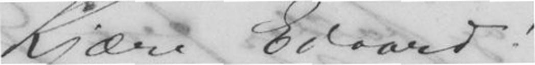Kjære Edvard!
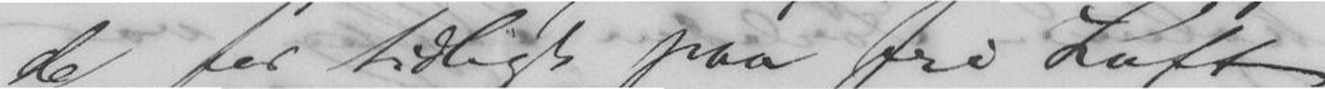de for tidligt paa fri Luft.
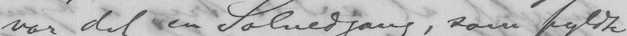var det en Solnedgang, som fyldte
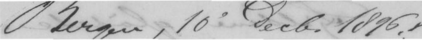Bergen, 10 Decbr. 1896!
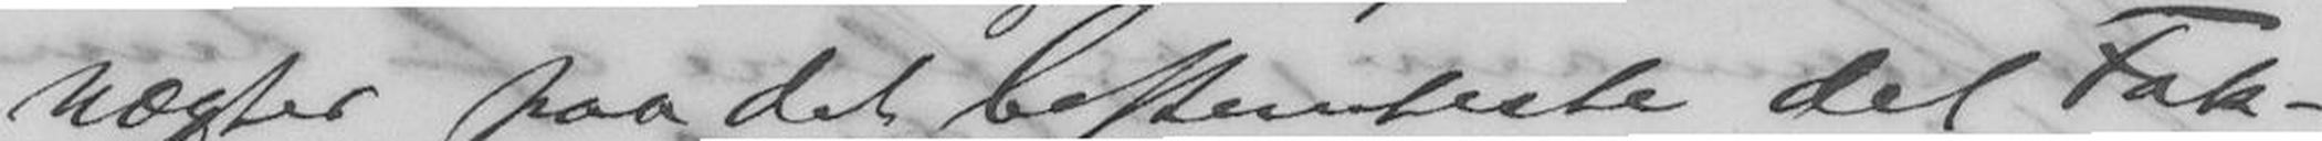nægter paa det bestemteste det Fak-
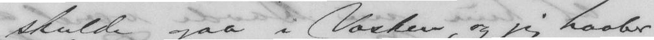skulde gaa i Vasken, og jeg haaber
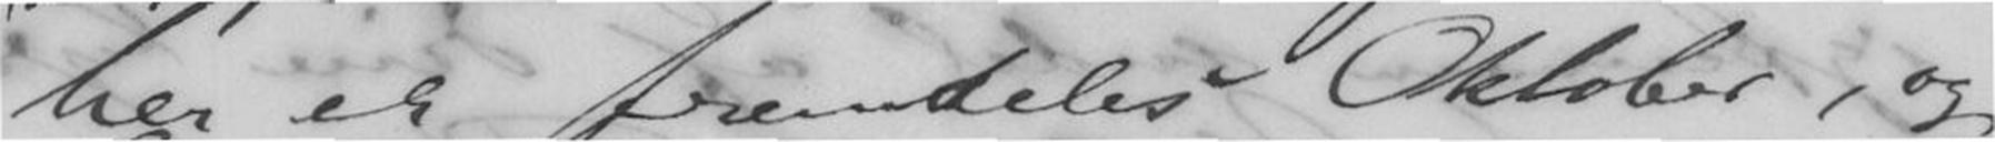her er fremdeles Oktober, og
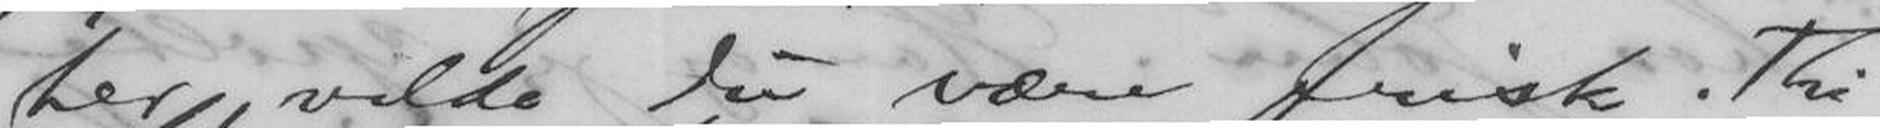her, vilde du være frisk. Thi
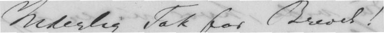Inderlig Tak for Brevet!
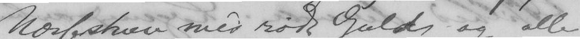Næsseskov med rødt Guld og alle
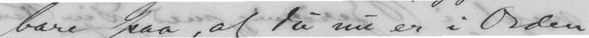bare paa, at du nu er i Orden
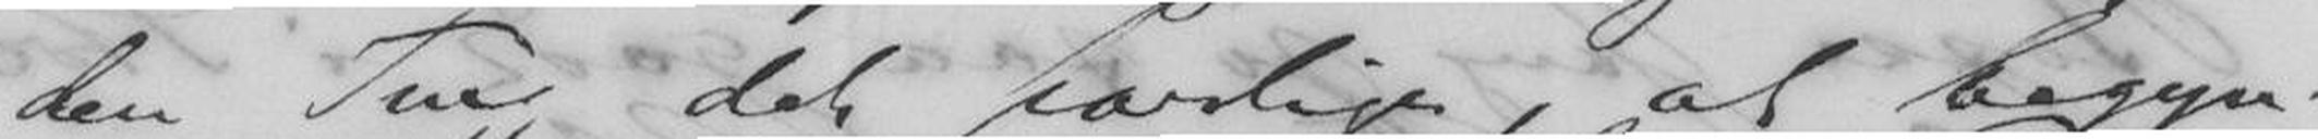den Ting det farlige, at begyn-
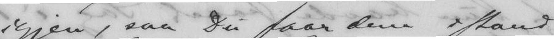igjen, saa Du faar dem istand
